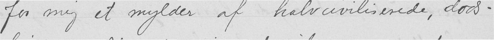for mig et mylder af halvciviliserede, døds-
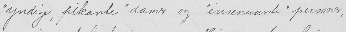"yndige, pikante" damer og "insenuante" personer,
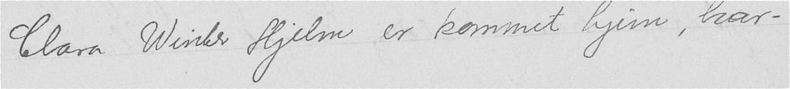Clara Winter Hjelm er kommet hjem, har-
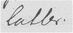latter.
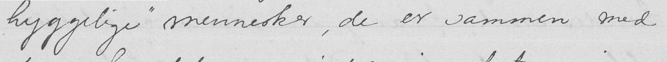hyggelige" mennesker, de er sammen med
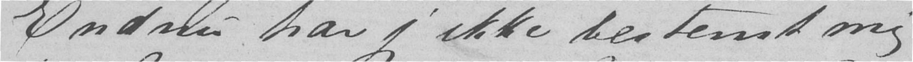Endnu har jeg ikke bestemt mig
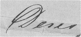Deres
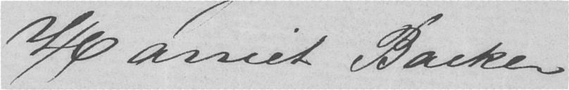Harriet Backer
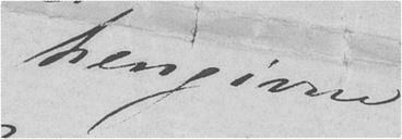hengivne
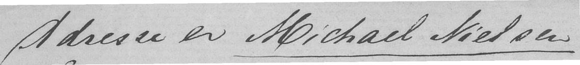Adresse er Michael Nielsen
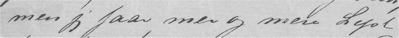men jeg faar mer og mere Lyst
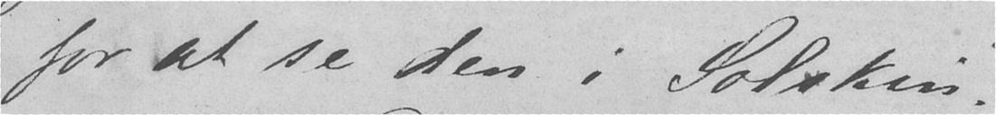for at se den i Solskin.
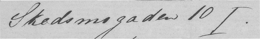Skedsmogaden 10 I.
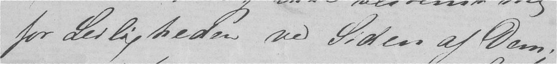for Leiligheden ved Siden af Dem;
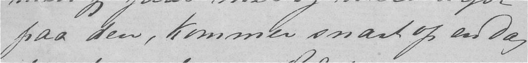paa den, kommer snart op en dag
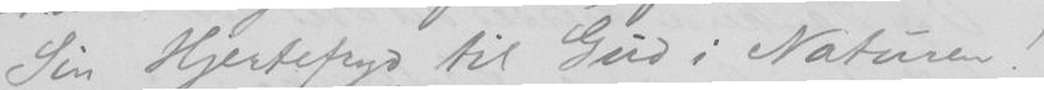Sin Hjertefryd til Gud i Naturen! -
